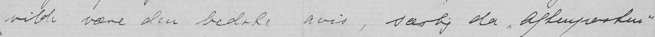vilde være den bedste hvis, særlig da Aftenposten-
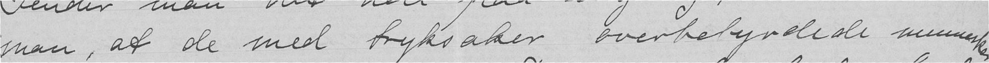man, at de med tryksaker overbebyrdede mennesker
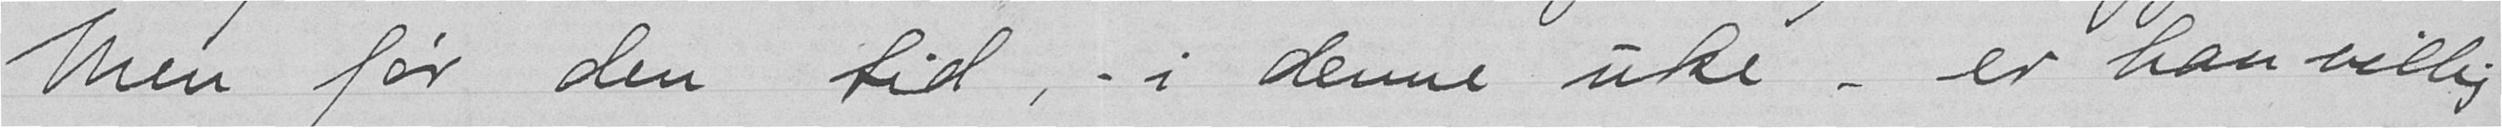Men før den tid, - i denne uke- er han villig
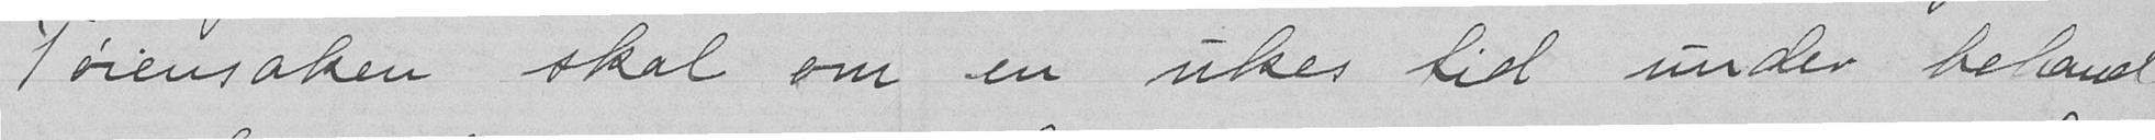Tøiensaken skal om en ukes tid under behand-
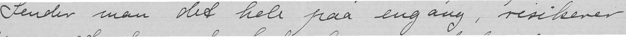Sender man det hele paa engang, risikerer
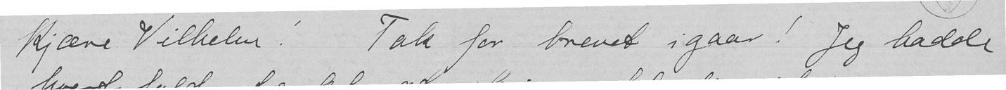Kjære Vilhelm. Tak for brevet igaar. Jeg hadde
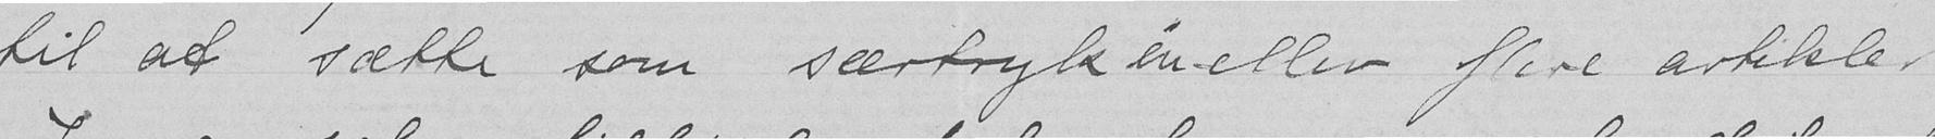til at sætte som særtryk en eller flere artikler.
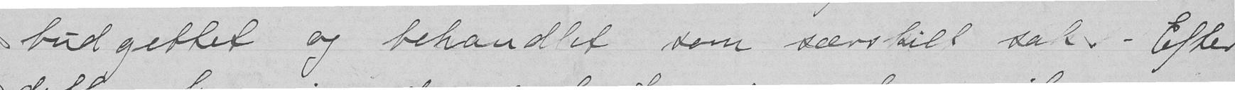budgettet og behandlet som særstilt sak - Efter
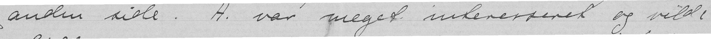anden side. H. var meget interesseret og vilde
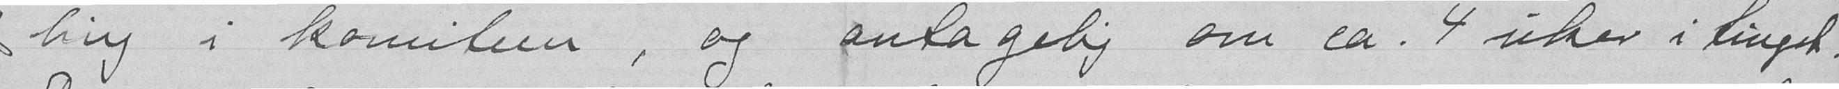ling i komiteen, og antagelig om ca. 4 uker i tinget.
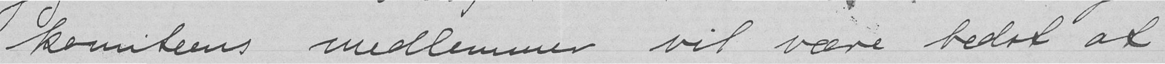komiteens medlemmer vil være bedse at
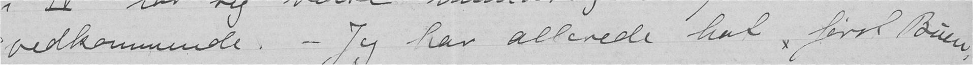vedkommende. - Jeg har allerede hat først Buen,
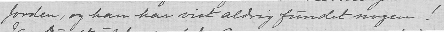Jorden, og han har vist aldrig fundet nogen!
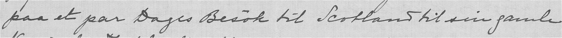paa et par Dages Besøk til Scotland til sin gamle
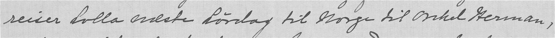reiser Lolla næste lørdag til Norge til Onkel Herman,
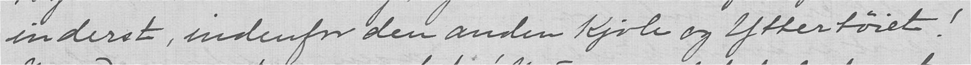inderst, indenfor den anden Kjole og Yttertøiet!
